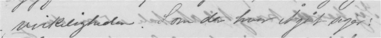virkeligheden. Som der hvor Ågåt siger:
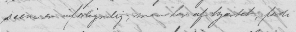scene er uforlignelig; man ler af hjærtet, fordi
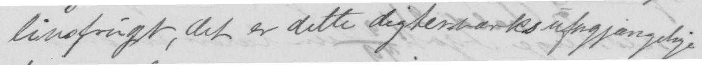livsfrugt, det er dette digterværks uforgjængelige
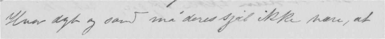Hvor dyb og sand må deres sjæl ikke være, at
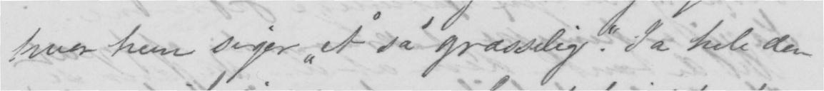hvor hun siger "å så græsselig". Ja hele den
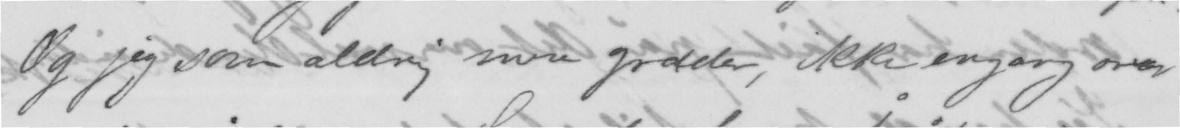Og jeg som aldrig mere græder, ikke engang over
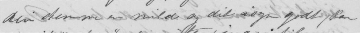din stemme er mild og dit åsyn godt, kan
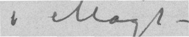i Magt –
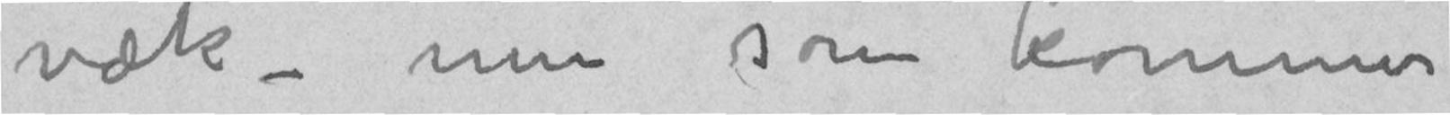væk – men som kommer
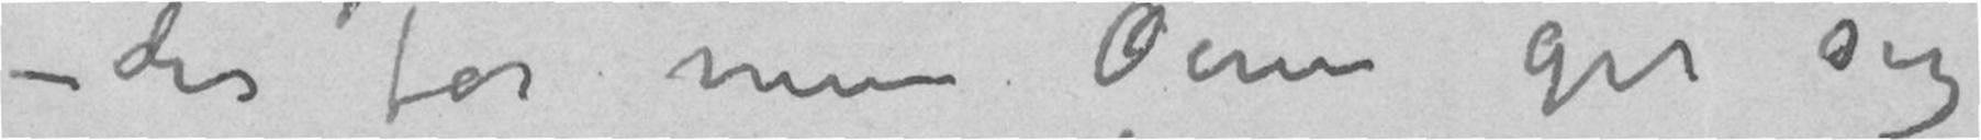– der for mine Øine gir sig
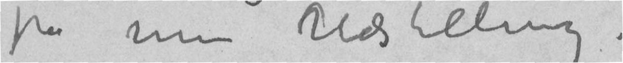på min Udstilling.
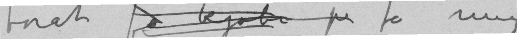forat få kjøbefå mig
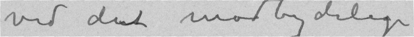ved den modbydelige
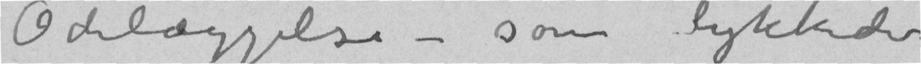Ødelæggelse – som lykkedes
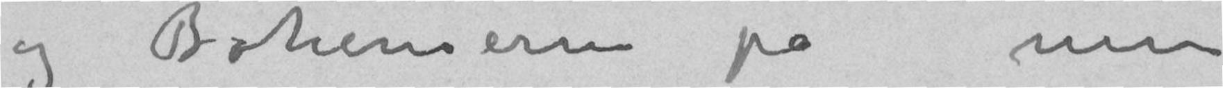og Bohemerne på min
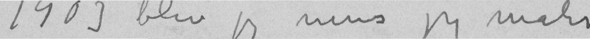1903	blev jeg mens jeg maler
